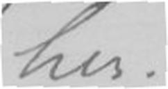her.
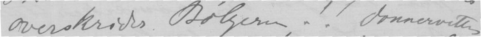overskrides Bølgeren!! Vonnervittig
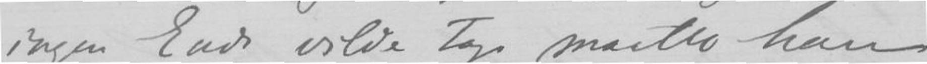ingen Ende vilde Tage maatte han
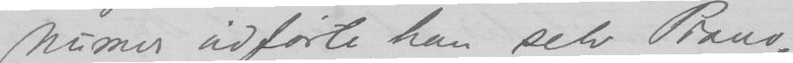Numer udførte han selv Piano-
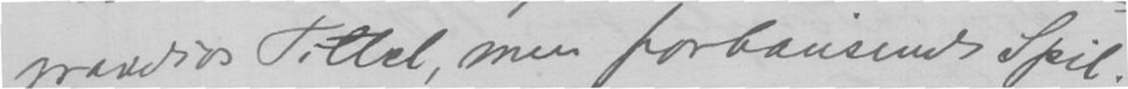grandios Tittal, men forbausende Spil.
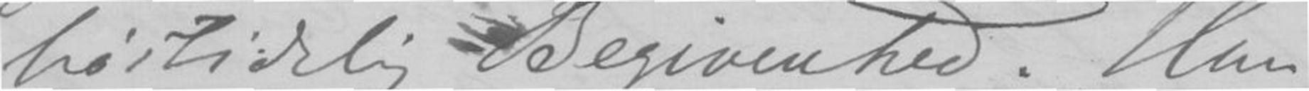høitidelig Begivenhed. Han
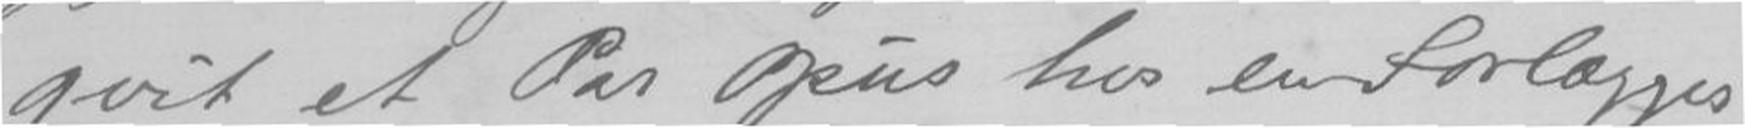qvit et Par Opus hos en Forlægger
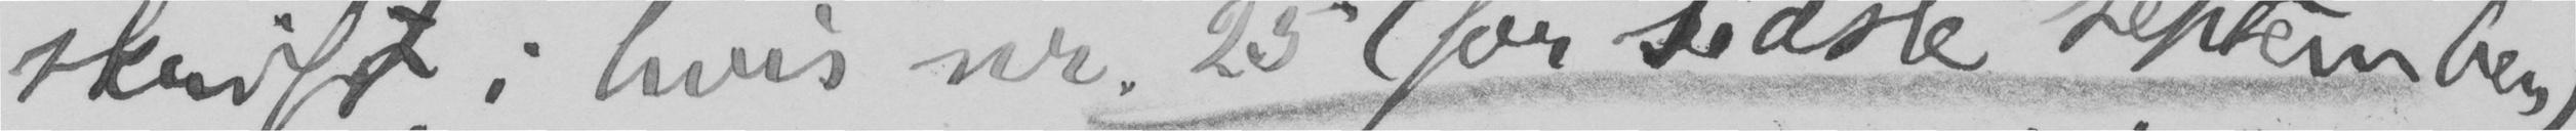skrift i hvis nr. 25 (for sidste september),
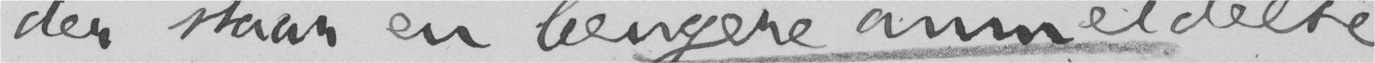der staar en længere anmeldelse
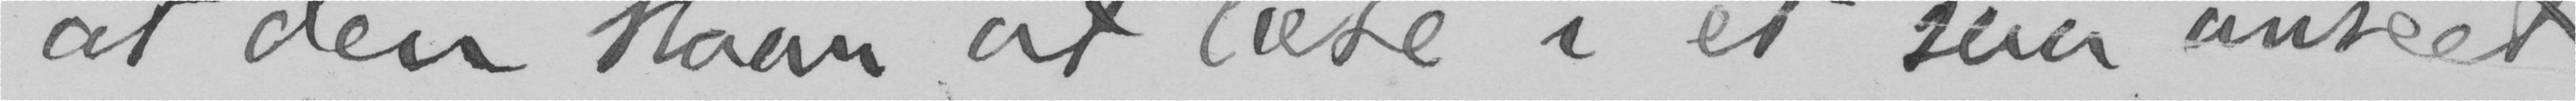at den staar at læse i et saa anseet
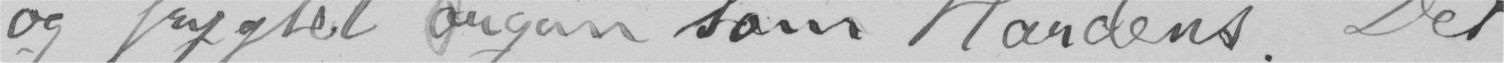og frygtet organ som Hardens. Det
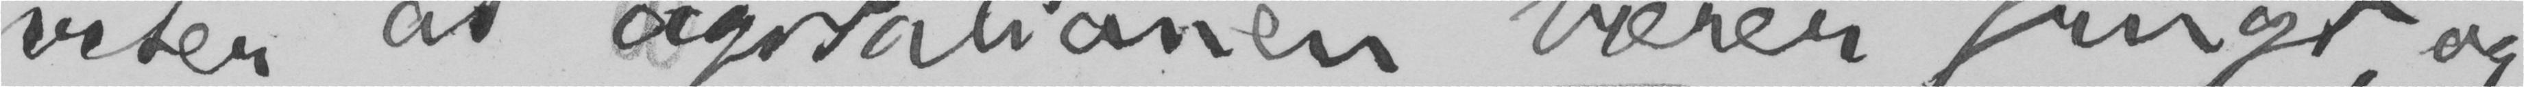viser, at agitationen bærer frugt, og
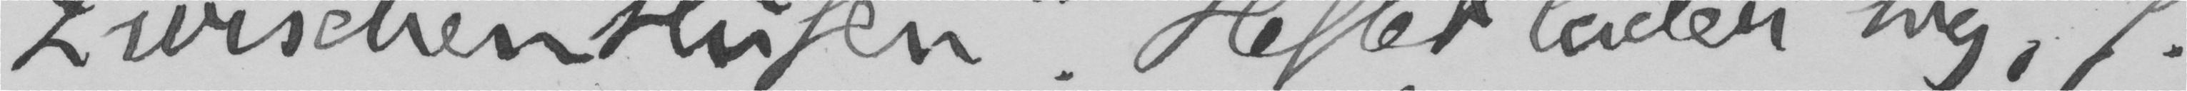Zwischenstufen». Heftet lader sig, f.
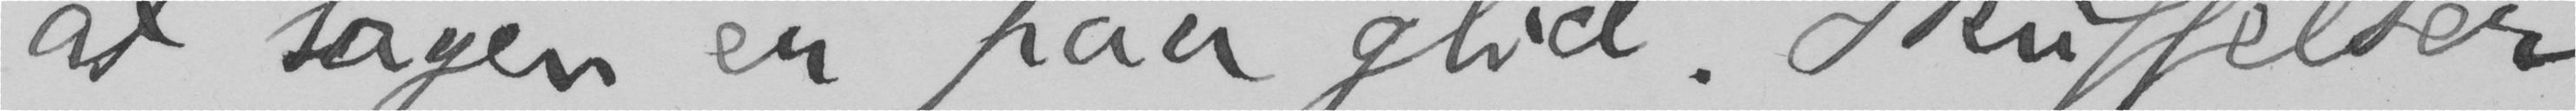at sagen er paa glid. Skuffelser
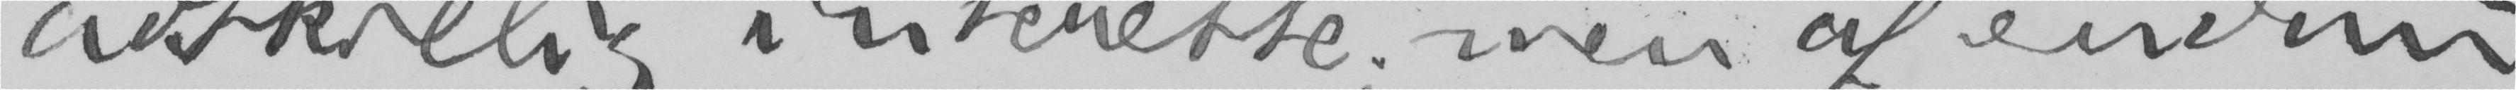adskillig interesse, men af endnu
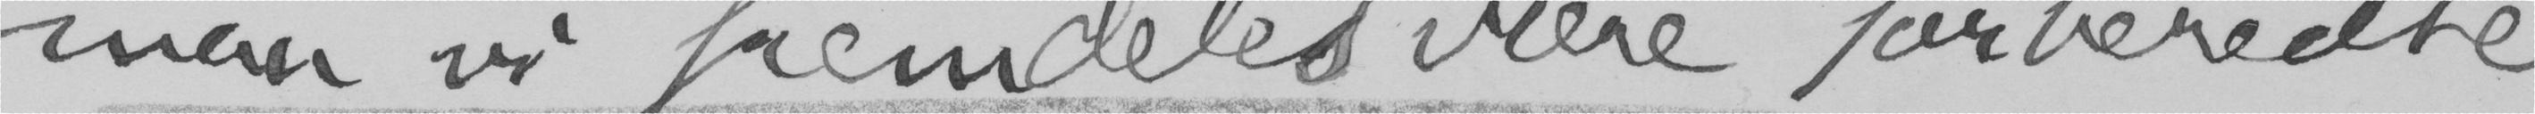maa vi fremdeles være forberedte
